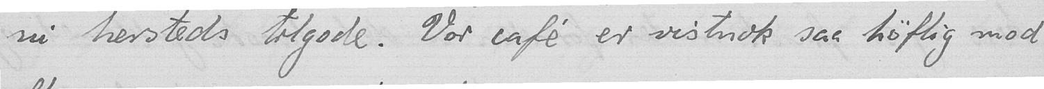ni hersteds tilgode. Vor café er vistnok saa høflig mod
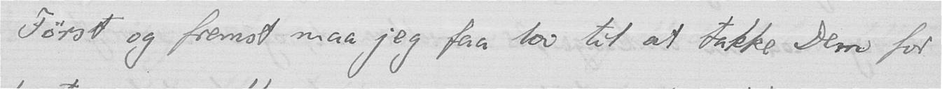Først og fremst maa jeg faa lov til at takke Dem for
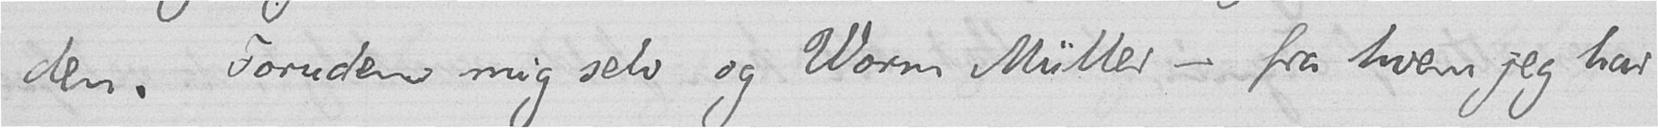den. Foruden mig selv og Worm Müller - fra hvem jeg har
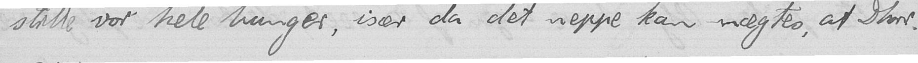stille vor hele hunger, især da det neppe kan nægtes, at Dhor-
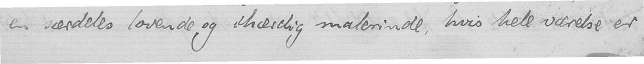en særdeles lovende og ihærdig malerinde, hvis hele værelse er
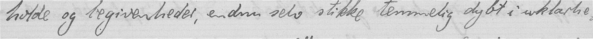holde og begivenheder, endnu selv stikke temmelig dybt i uklarhe-
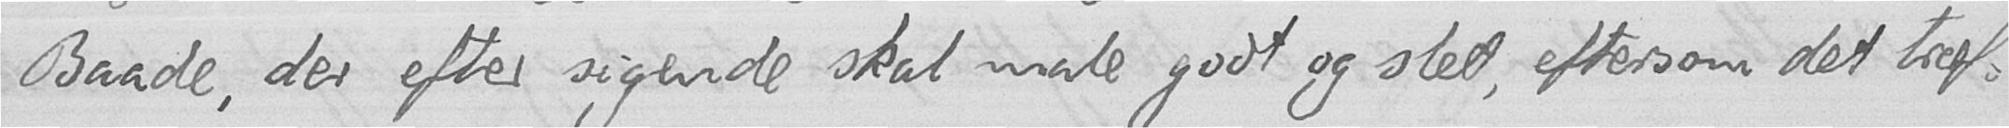Baade, der efter sigende skal male godt og slet, eftersom det træf-
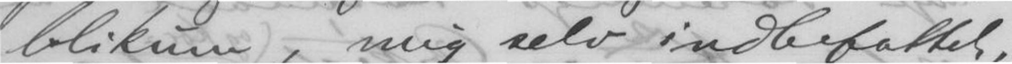blikum, mig selv indbefattet,
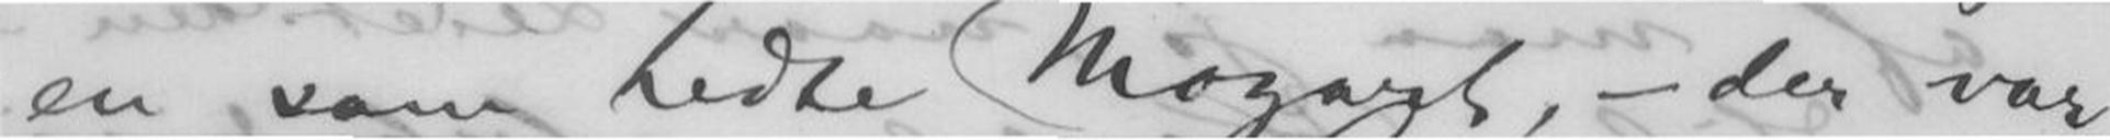en som hedte Mozart, - der var
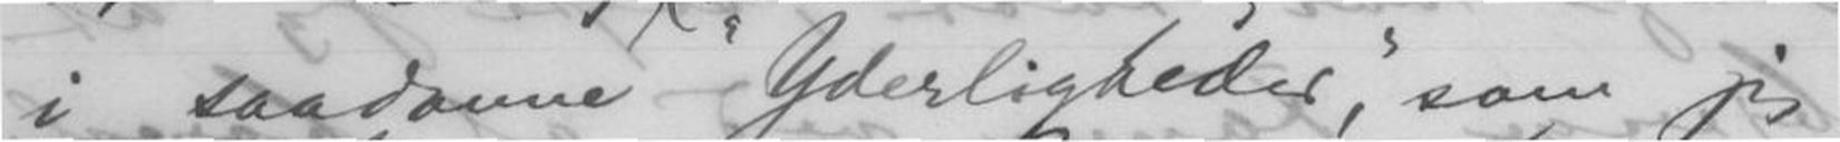i saadanne "Yderligheder", som jeg
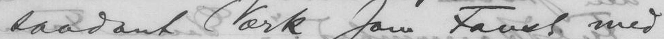saadant Værk som Faust med
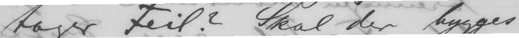tager Feil? Skal der bygges
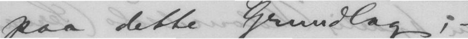paa dette Grundlag; -
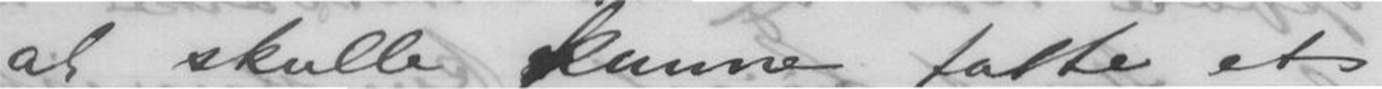at skulle kunne fatte et
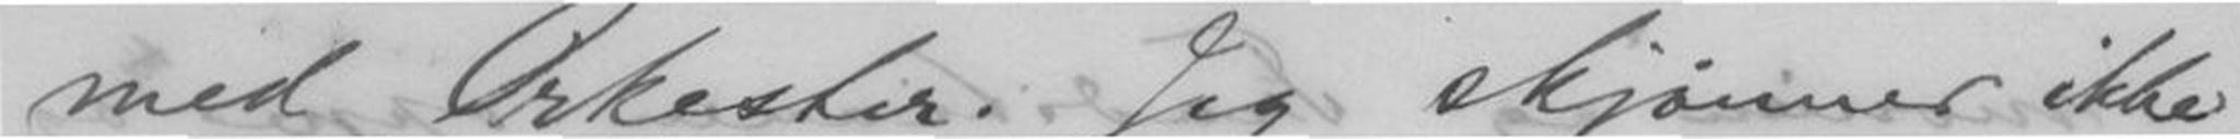med Orkester. Jeg skjønner ikke
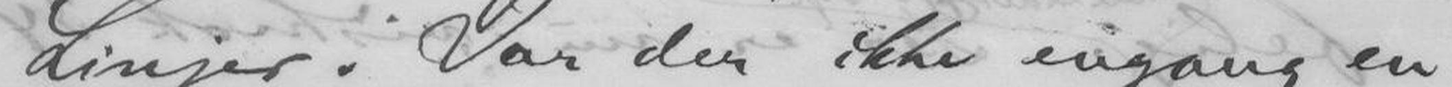Linjer. Var der ikke engang en
